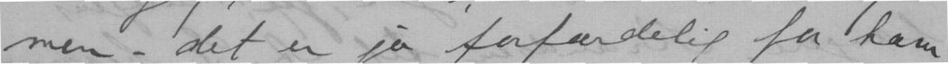men - det er jo forfædelig for ham.
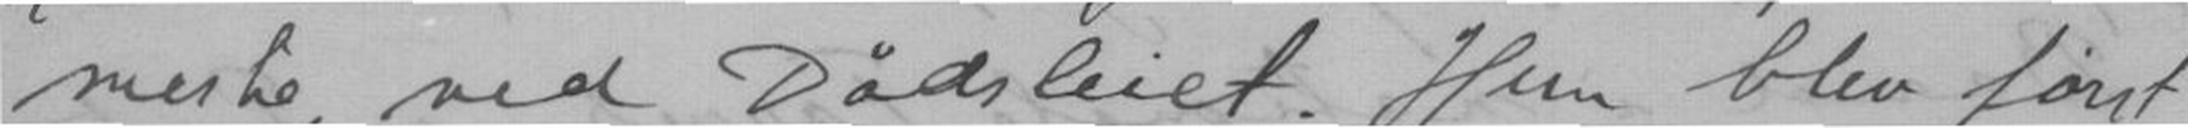meste, ved Dødsleiet. Hun blev først
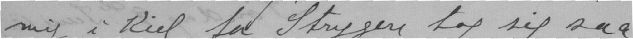mig i Kiel, for Strygere tog sig saa
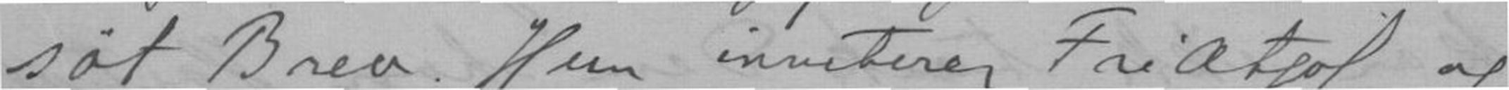søt Brev. Hun inviterer Fridtjof og
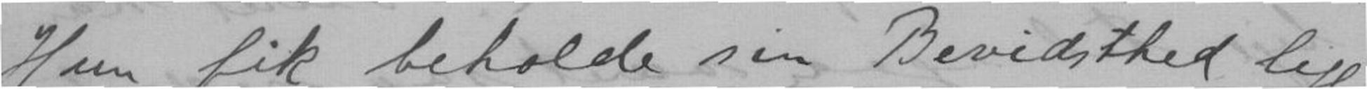Hun fik beholde sin Bevidshed lige
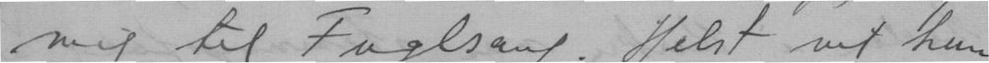mig til Fuglsang. Helst vil hun
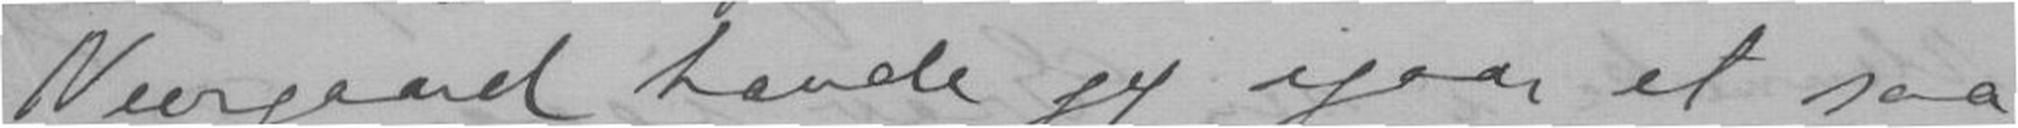Neergaard havde jeg igaar et saa
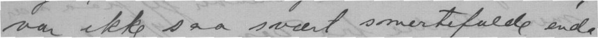var ikke saa svært smertefulde enda.
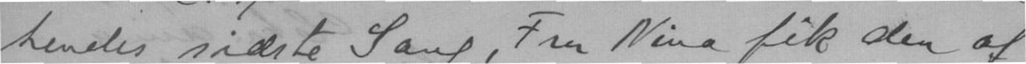hendes sidste Sang, Fru Nina fik den af
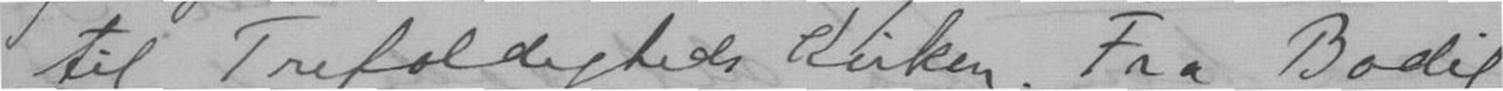til Trefoldighets Kirken. Fra Bodil
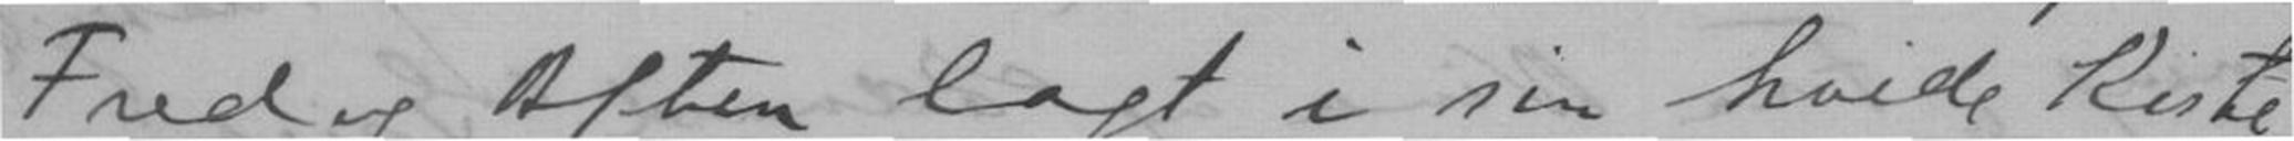Fredag Aften lagt i sin hvide Kiste
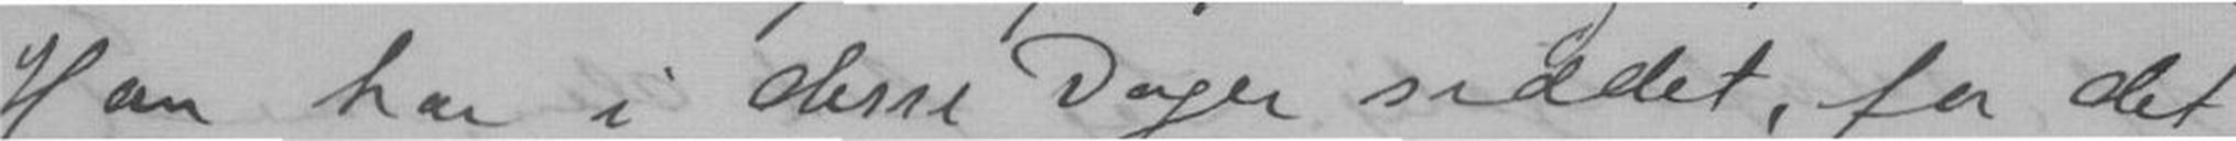Han har i disse Dager siddet, for det
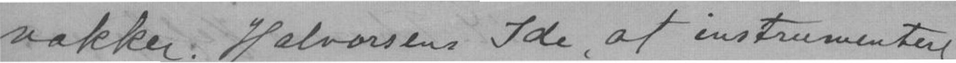vakker. Halvorsens Ide, at instrumentere
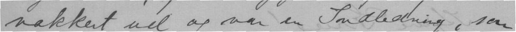vakkert ud og var en Indledning, som
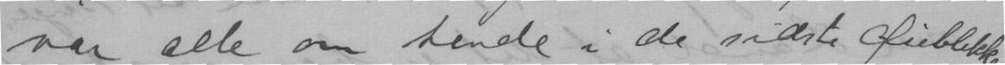var alle om hende i de sidste Øieblikke.
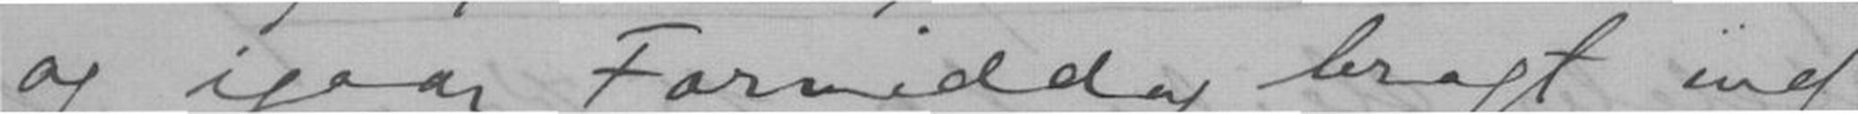og igaar Formiddag bragt ind
